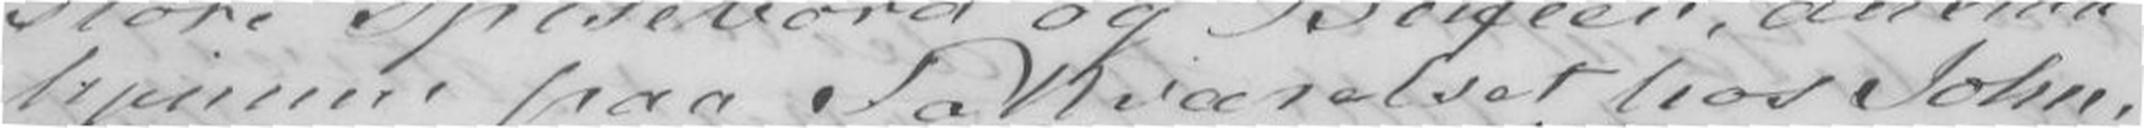hjemme paa Takværelset hos John,
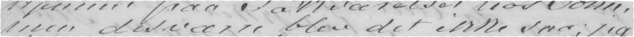men desværre blev det kke saa; jeg,
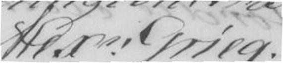Alexxr Grieg.
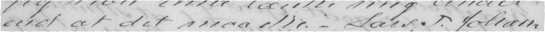end at det maa ske. - Lars T. Johan-
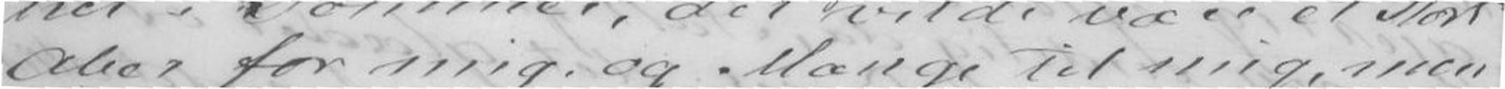Aber for mig, og Mange til mig, men
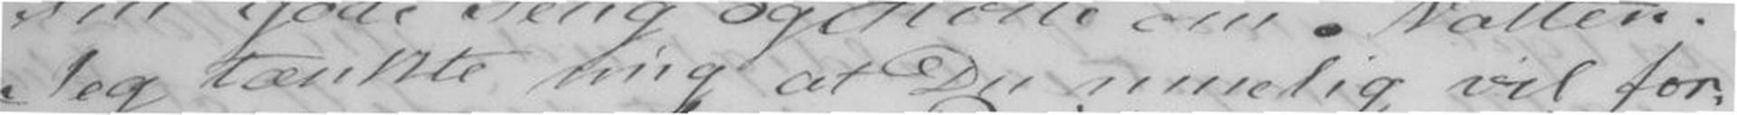Jeg tænkte mig at Du muelig vil for-
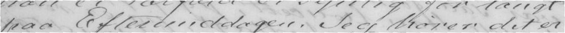paa Eftermiddagen. Jeg hører det er
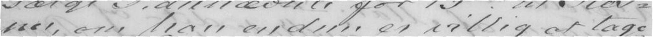ner, om han endnu er villig at tage
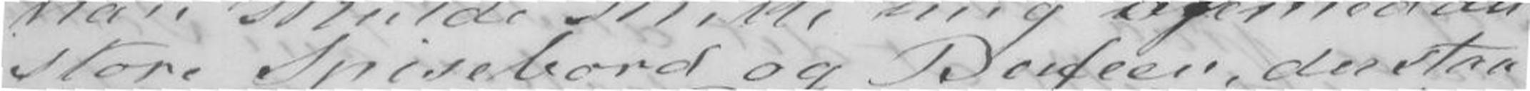store Spisebord og Bufeer, der staa
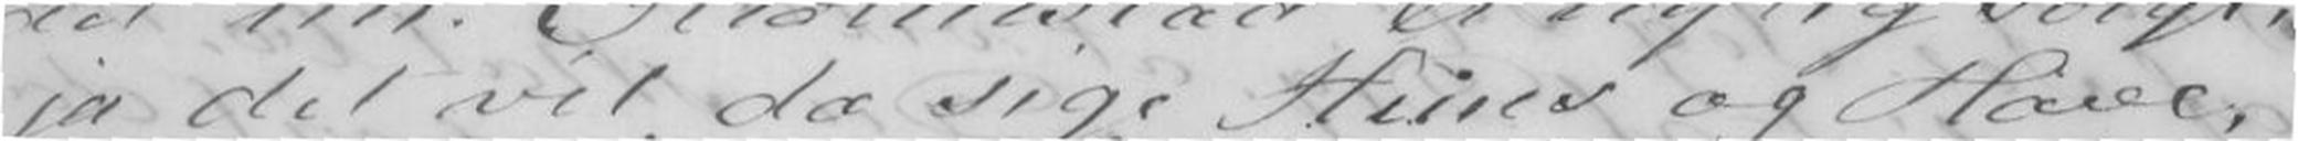ja det vil da sige Huus og Have,
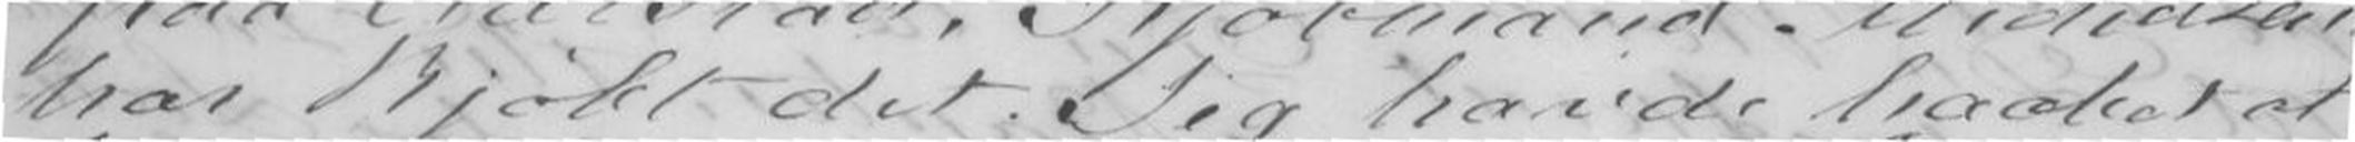har kjøbt det. Jeg havde haabet at
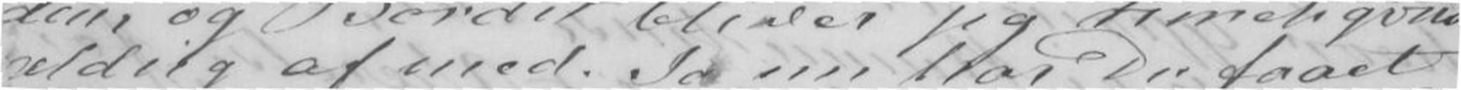aldrig af med. Ja nu har Du faaet
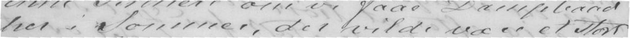her i Sommer, der vilde være et stort
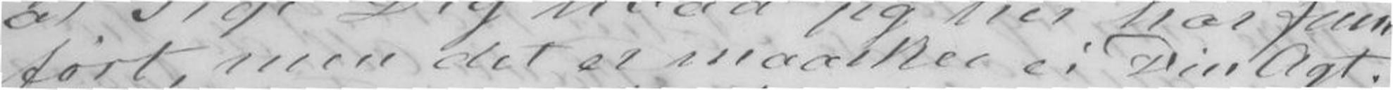ført, men det er maaskee ei Din Agt.
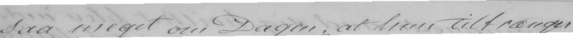saa meget om Dagen, at hun tiltrænger
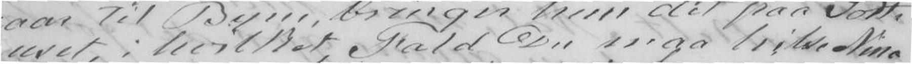user, i hvilket Fald Du maa hilse Nina
